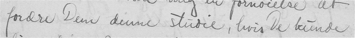fordre Dem denne studie, hvis de kunde
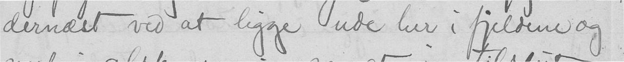dernæst ved at ligge lude her i fjeldene og
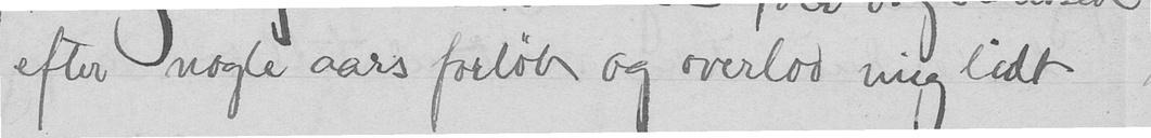efter nogle aars forløb og overlod mig lidt
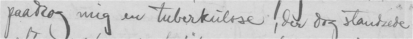paadrog mig en tuberkulose, ver dog standsede
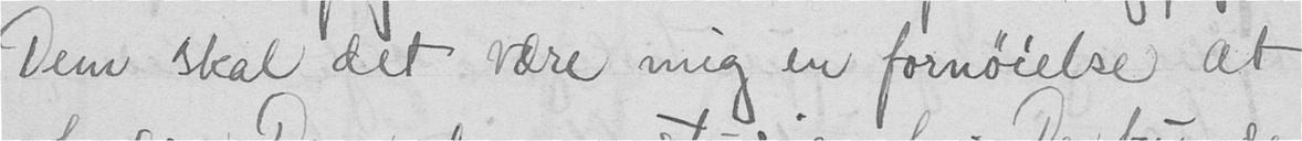Dem skal det være mig en fornøielse, at
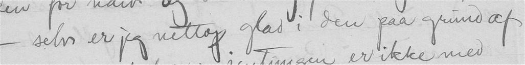- selv er jeg nettop glad i den paa grund af
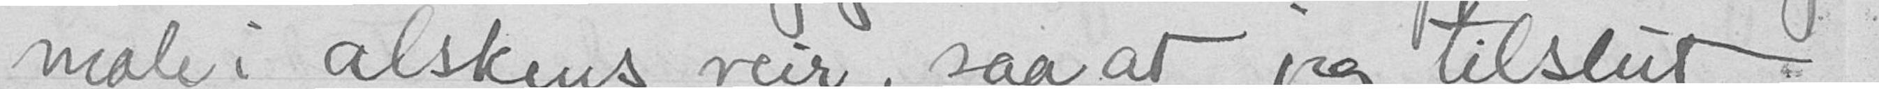male i alskens veir, saa at jeg tilslut
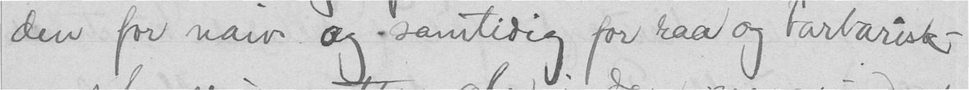den for naiv og samtidig for raa og barbarisk-
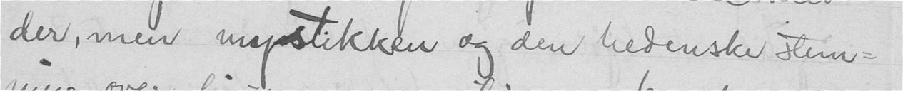der, men mystikken og den hedenske stem-
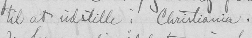til at udstille i Christiania.
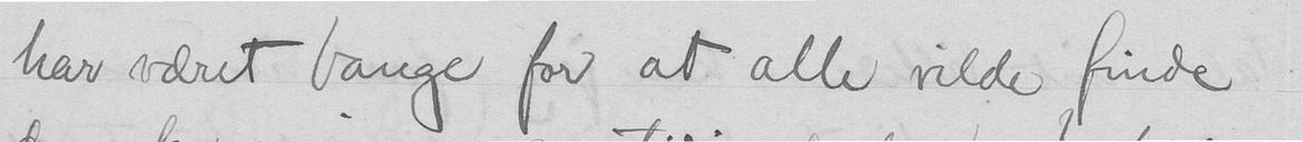har været bange for at alle vilde finde
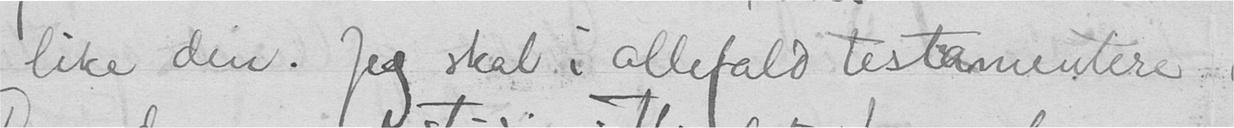like den. Jeg skal i allefald testamentere
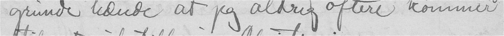grunde hænde at jeg aldrig oftere kommer
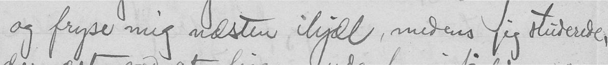og fryse mig næsten ihjel, medens jeg studerede
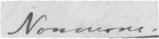Nonnerne.
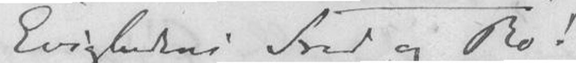Evighedens Fred og Ro!
